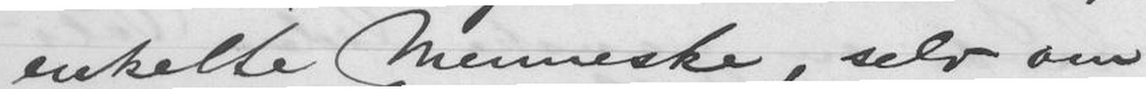enkelte Menneske, selv om
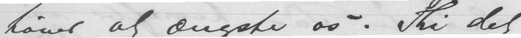høver at ængste os. Thi det
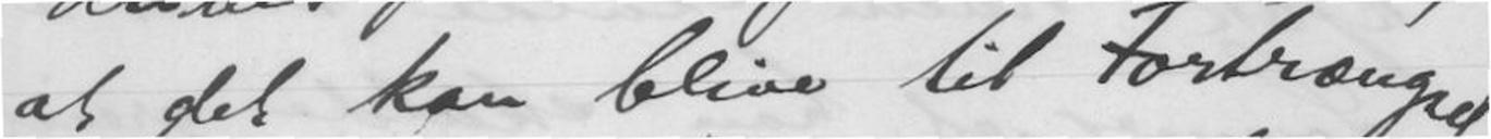at det kan blive til Fortrængsel
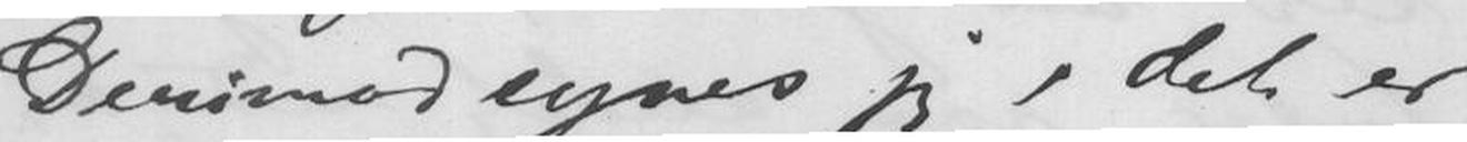Derimod synes jeg, det er
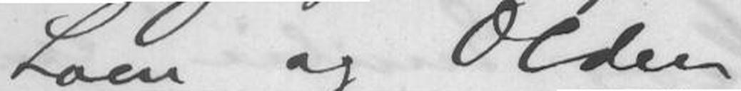Loen og Olden!
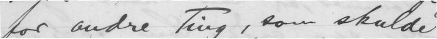for andre Ting, som skulde
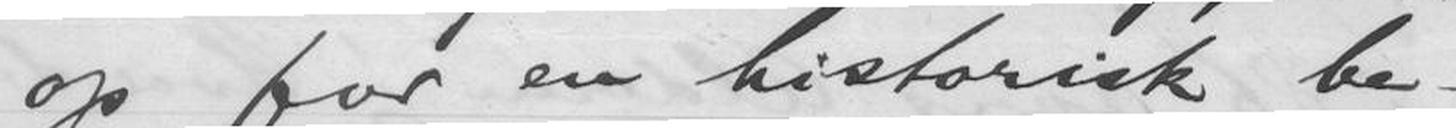op for en historisk be-
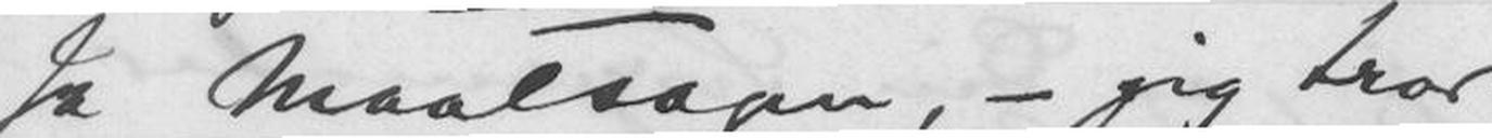Ja Maalsagen, - jeg tror
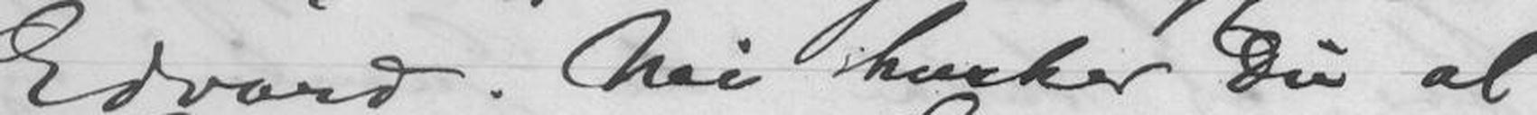Edvard. Nei husker Du al
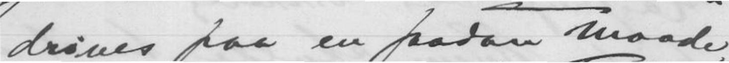drives paa en saadan Maade,
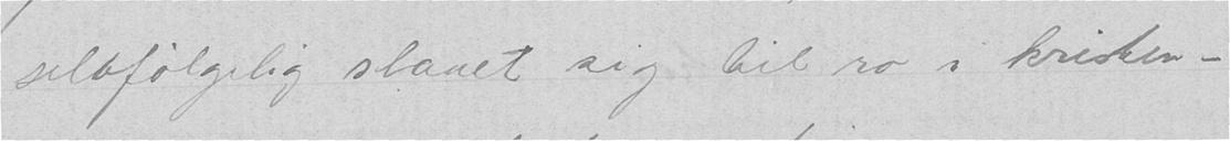selvfølgelig slaaet sig til ro i kristen-
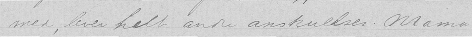med, lever helt andre anskuelser. Mama
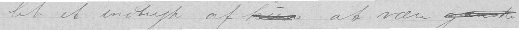let et indtryk af kun at være ganske
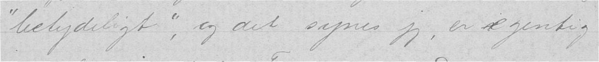"betydeligt", og det synes jeg, er egentlig
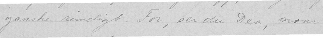ganske rimeligt. For, ser du Dea, naar
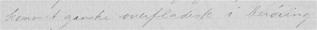kommet ganske overfladisk i berøring
